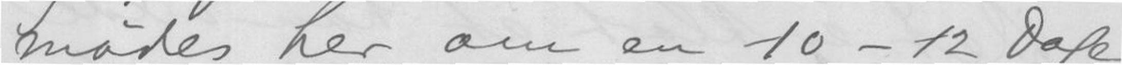mødes her om en 10 - 12 Dage.
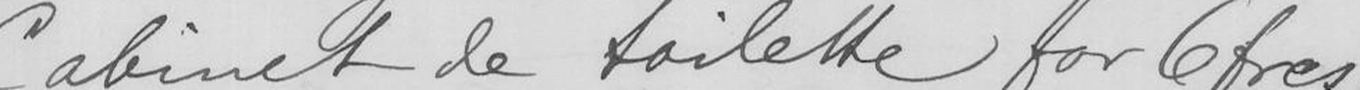Cabinet de toilette for 6 fres
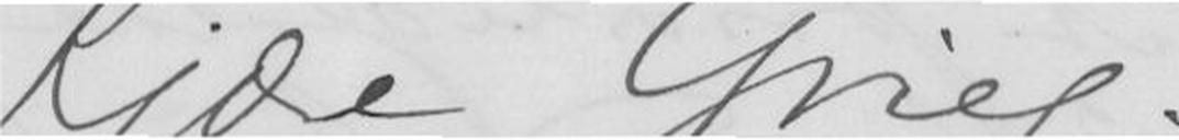Kjære Grieg
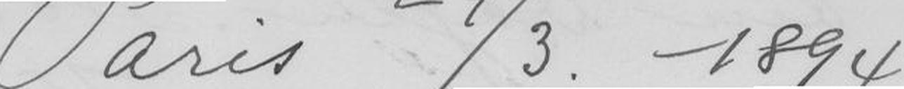Paris 29/3. 1894
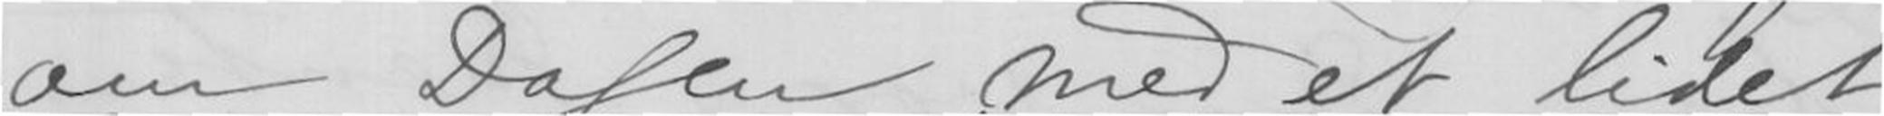om Dagen med et lidet
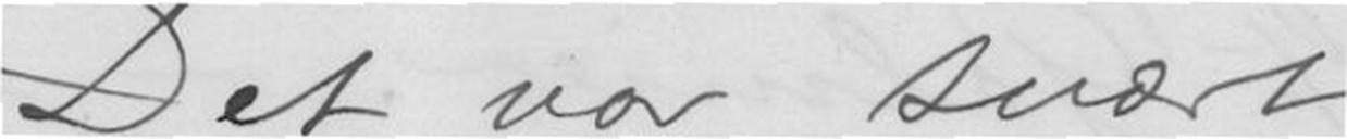Det var svært
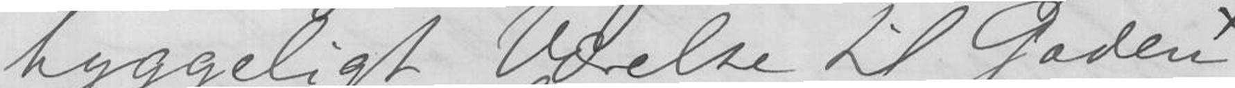hyggeligt Værelse til Gadenx
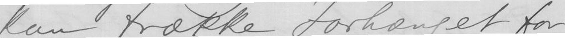kan trække Forhænget for
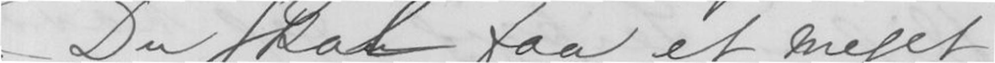Du skal faa et meget
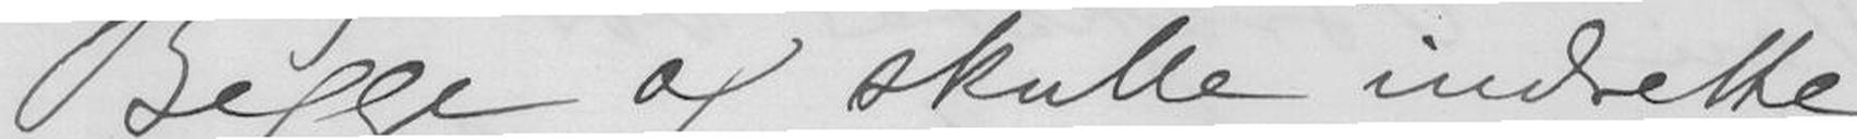Begge og skulle indrette
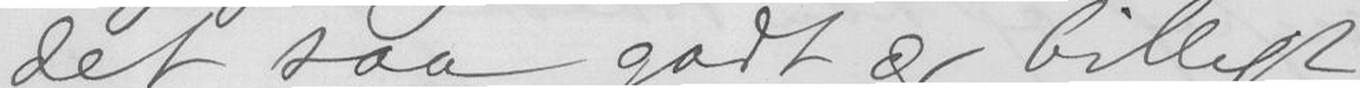det saa godt og billigt
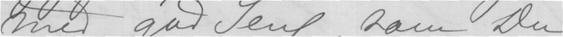med god Seng, som Du
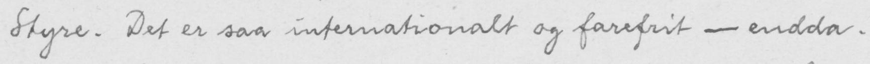Styre. Det er saa internationalt og farefrit - endda.
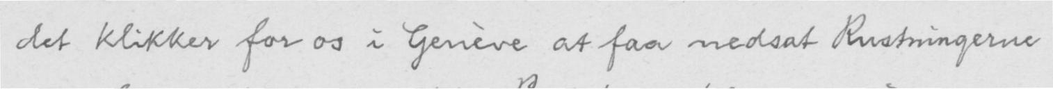det klikker for os i Genève at faa nedsat Rustningerne
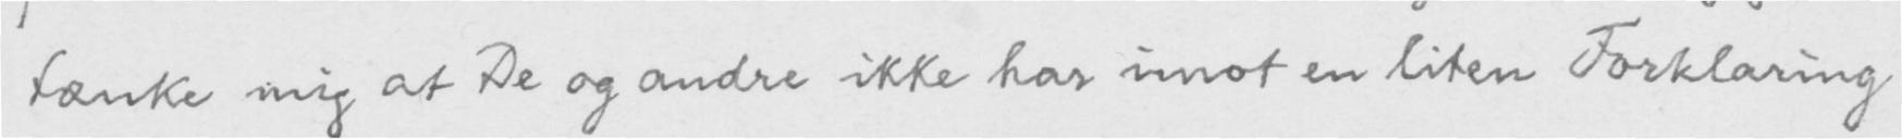tænke mig at De og andre ikke har imot en liten Forklaring
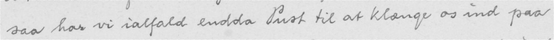saa har vi ialfald endda Pust til at klænge os ind paa
